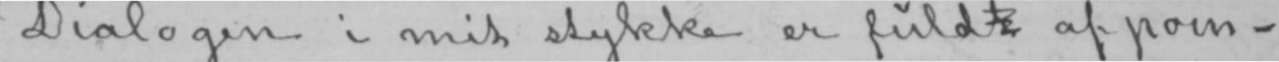Dialogen i mit stykke er fuldt af poin-
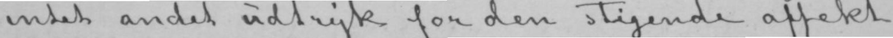intet andet udtryk for den stigende affekt,
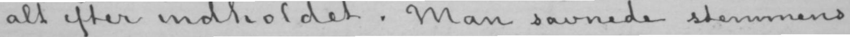alt efter indholdet. Man savnede stemmens
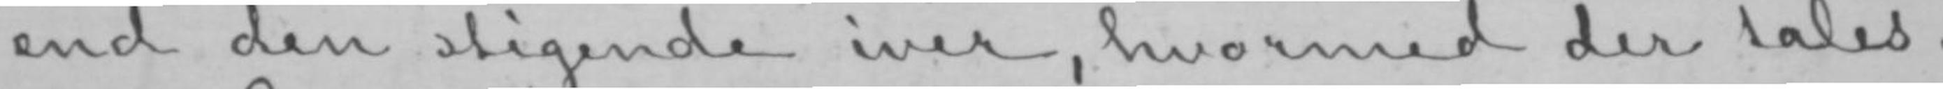end den stigende iver, hvormed der tales.
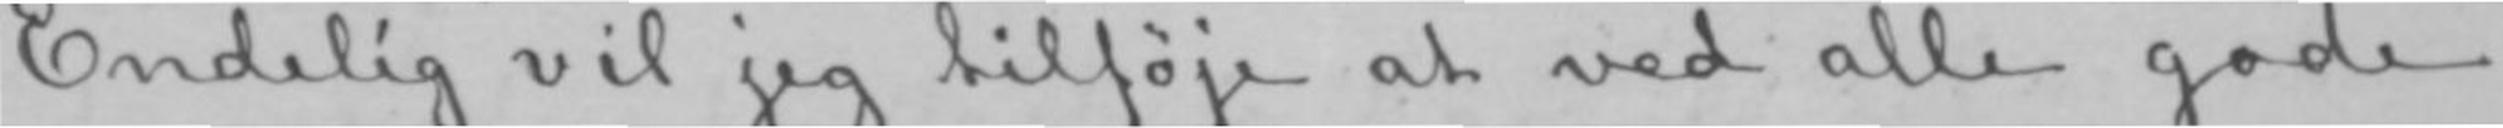Endelig vil jeg tilføje at ved alle gode
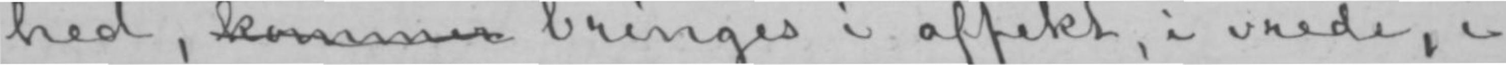hed, kommer bringes i affekt, i vrede, i
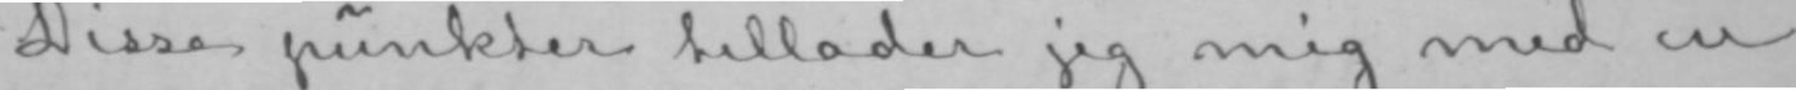Disse punkter tillader jeg mig med en
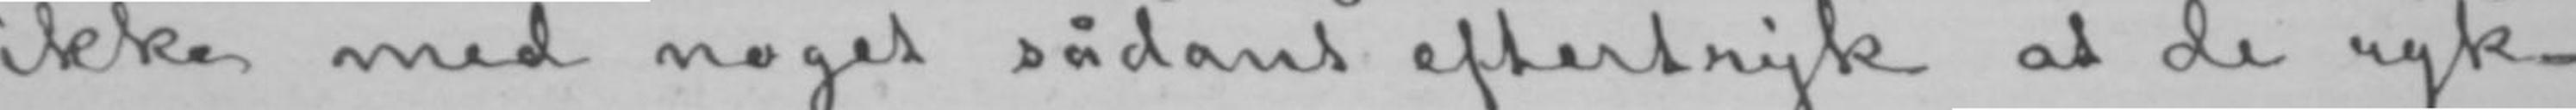ikke med noget sådant eftertryk at de ryk-
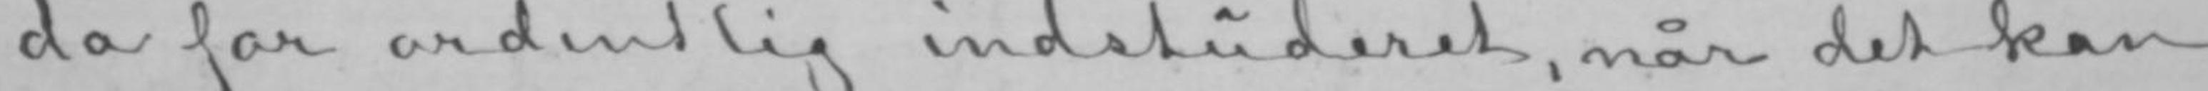da for ordentlig indstuderet, når det kan
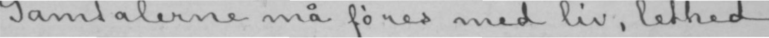Samtalerne må føres med liv, lethed
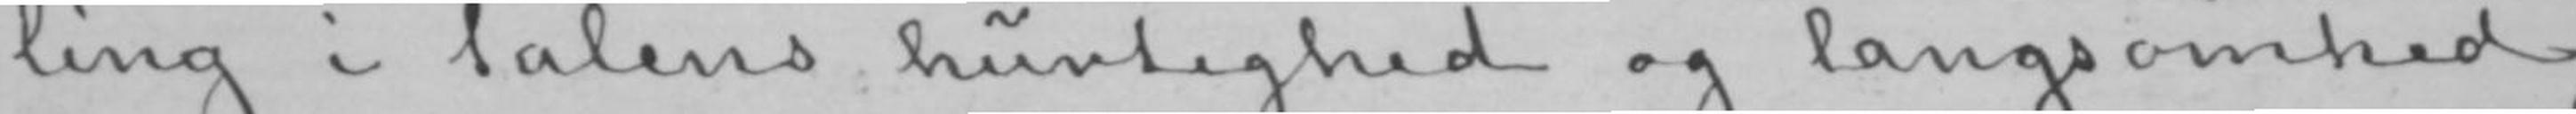ling i talens hurtighed og langsomhed,
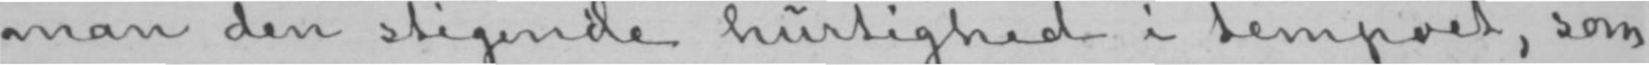man den stigende hurtighed i tempoet, som
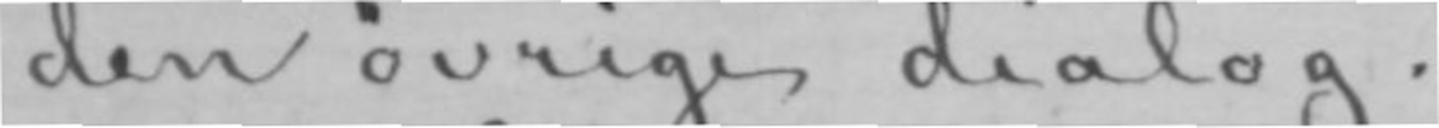den øvrige dialog.
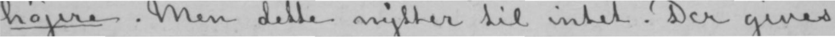højere. Men dette nytter til intet. Der gives
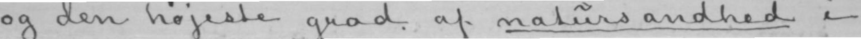og den højeste grad af natursandhed i
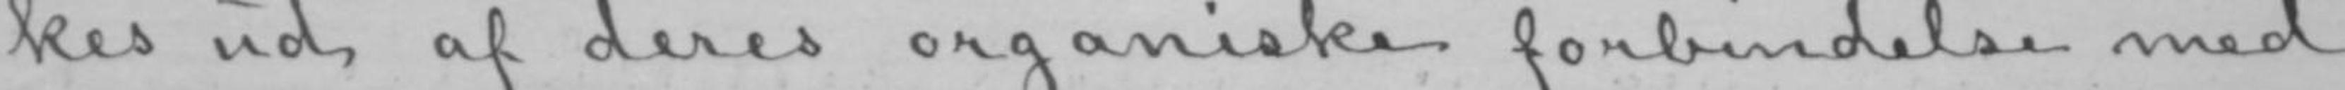kes ud af deres organiske forbindelse med
